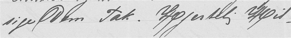sige Dem Tak. Hjertelig Hil-
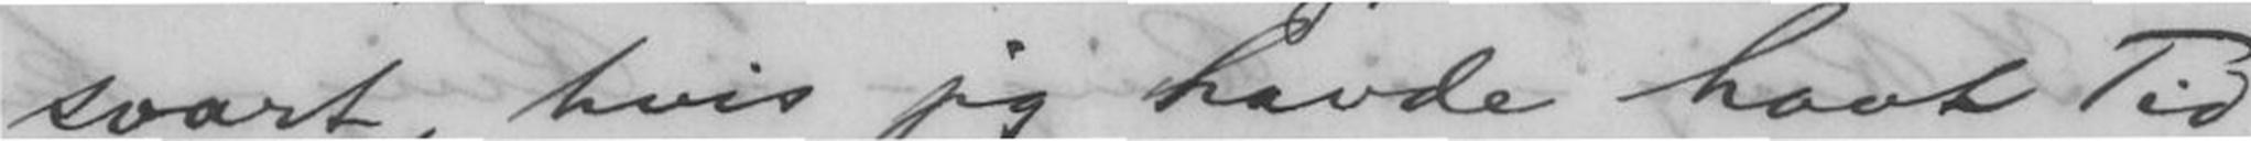svart, hvis jeg havde havt Tid
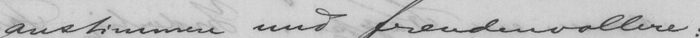anstimmen und freudenvollere:
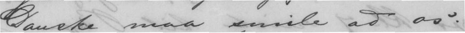Danske maa smile ad os.
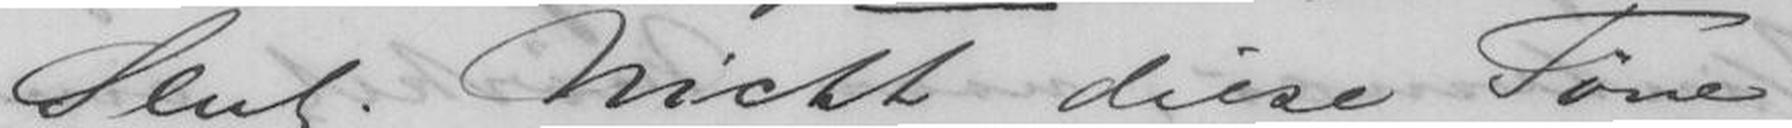Slut. Nicht diese Töne,
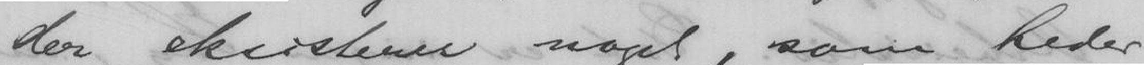der eksisterer noget, som heder
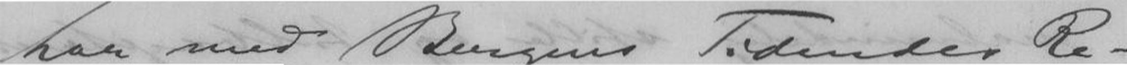har med Bergens Tidendes Re-
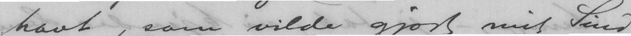havt, som vilde gjort mit Sind
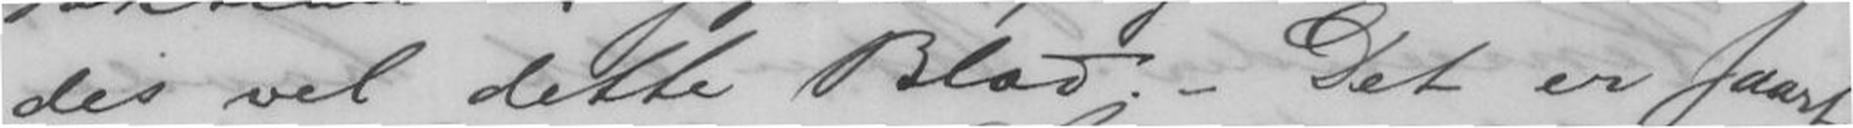des vel dette Blad. - Det er saart
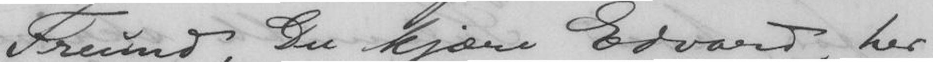Freund, Du kjære Edvard, her
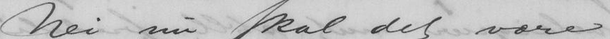Nei nu skal det være
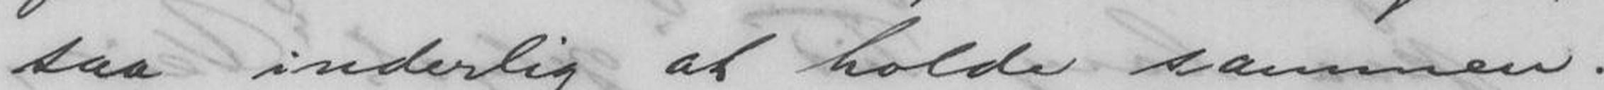saa inderlig at holde sammen.
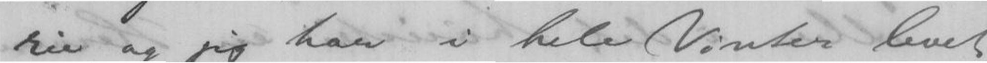rie og jeg har i hele Vinter levet
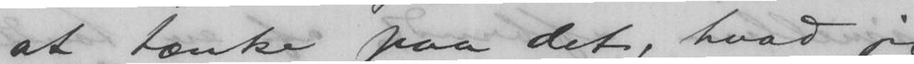at tænke paa det, hvad jeg
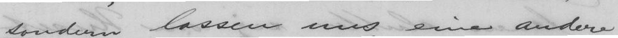sondern lassen uns eine audere
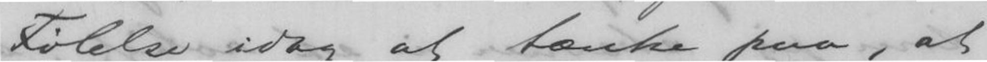Følelse idag at tænke paa, at
